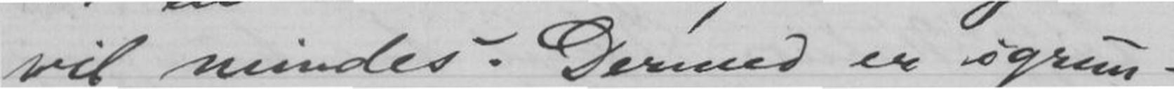vil mindes. Dermed er igrun-
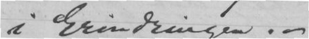i Erindringen. -
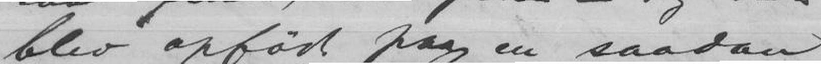blev opført paa en saadan
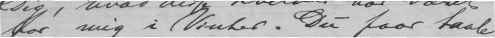for mig i Vinter. Du faar taale
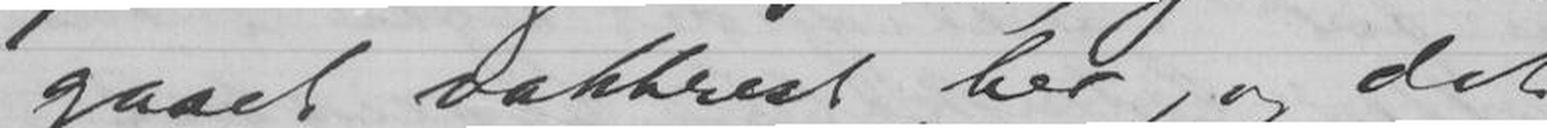gaaet vakkrest her, og det
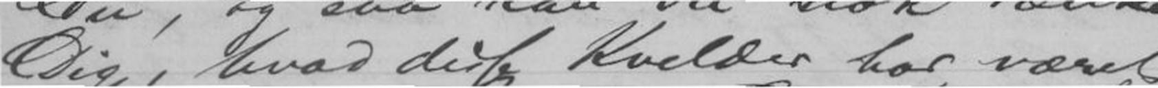Dig, hvad disse Kvelder har været
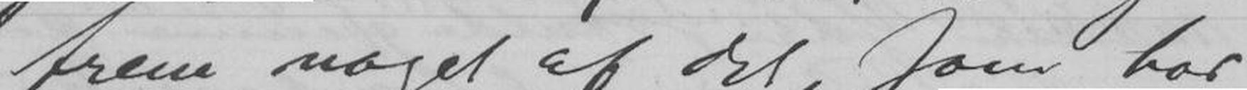frem noget af det, som har
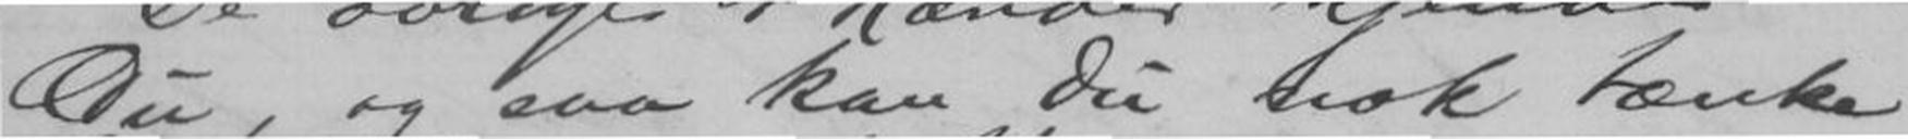Du, og saa kan Du nok tænke
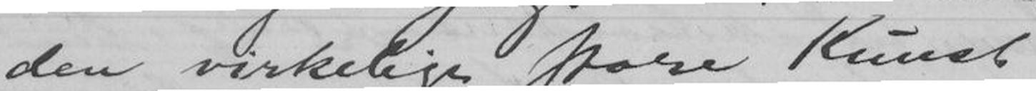den virkelige store Kunst
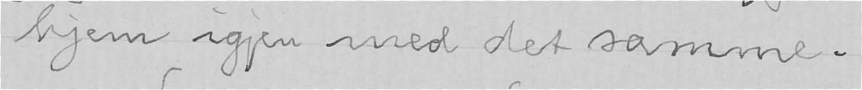hjem igjen med det samme.
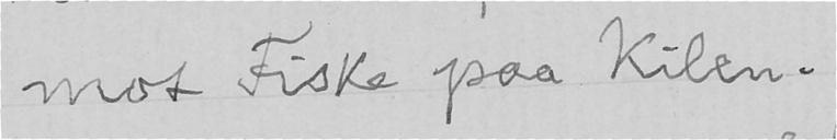mot Fiske paa Kilen.
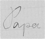Papa
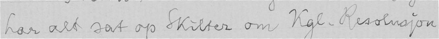har alt sat op Skilter om Kgl. Resolusjon
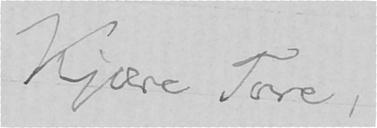Kjære Tore,
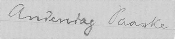Andendag Paaske
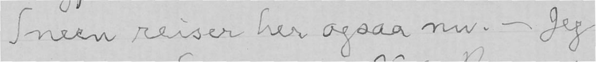Sneen reiser her ogsaa nu. - Jeg
